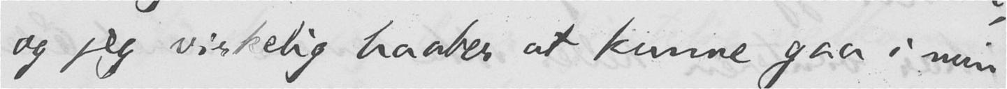og jeg virkelig haaber at kunne gaa i min
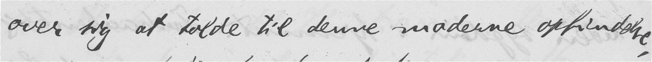over sig at tolde til denne moderne opfindelse,
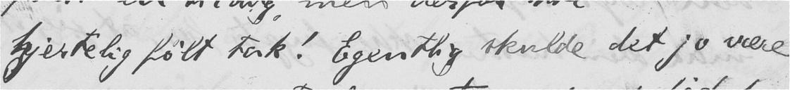hjertelig følt tak! Egentlig skulde det jo være
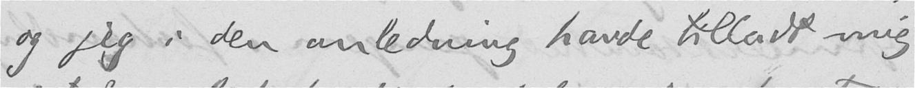og jeg i den anledning havde tilladt mig
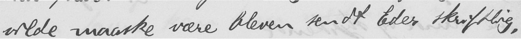vilde maaske være bleven sendt Eder skriftlig,
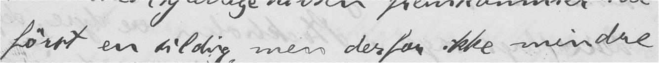først en sildig, men derfor ikke mindre
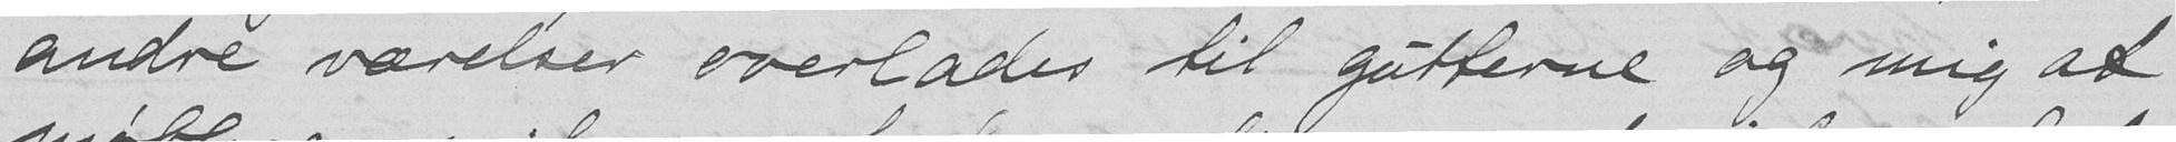andre værelser overlades til gutterne og mig at
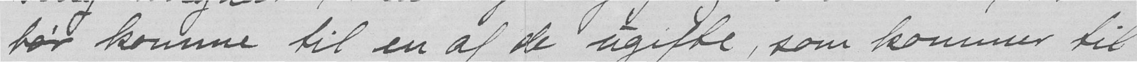bør komme til en af de ugifte, som kommer til
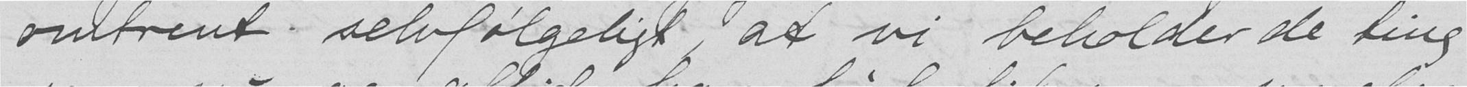omtrent selvfølgeligt at vi beholder de ting
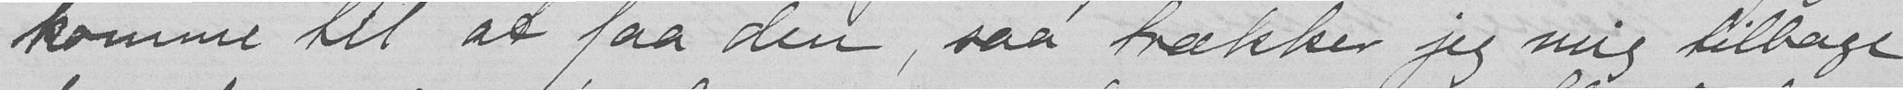komme til at faa den, saa trækker jeg mig tilbage
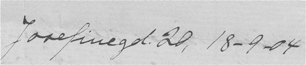Josefinegd. 20, 18-9-04
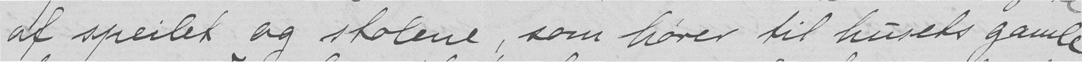af speilet og stolene som hører til husets gamle
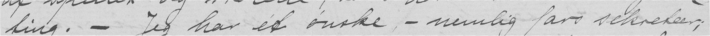ting. - Jeg har et ønske, - nemlig fars sekretær;
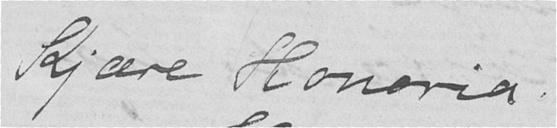Kjære Honoria!
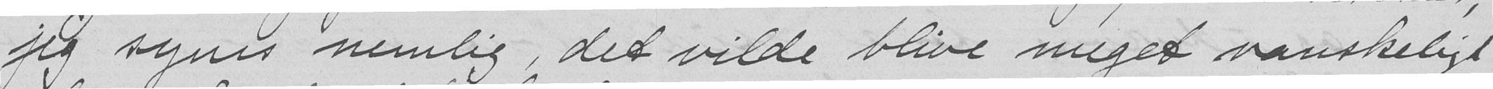jeg synes nemlig, det vilde blive meget vanskeligt
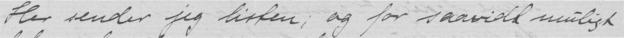Her sender jeg listen; og for saavidt muligt
